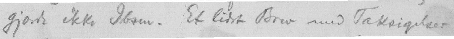gjorde ikke Ibsen. Et lidet Brev med Taksigelser
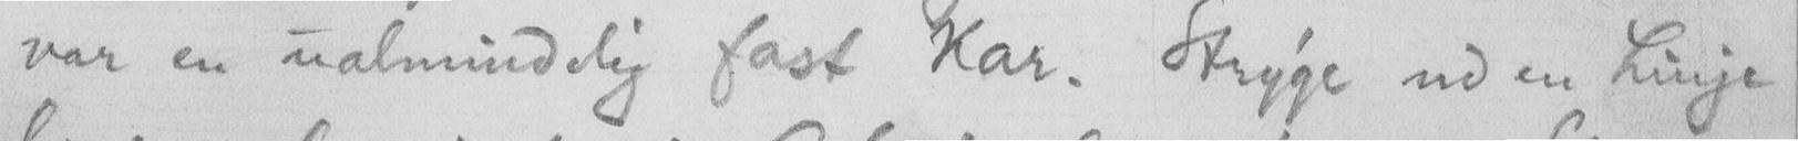var en ualmindelig fast Kar. Stryge ud en Linje
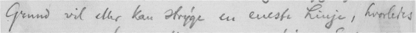Grund vil eller kan stryge en eneste Linje, hvorledes
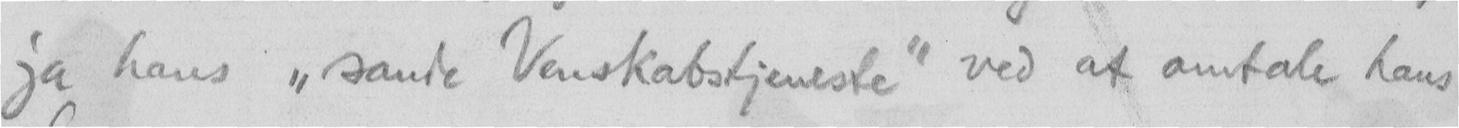ja hans "sande Venskabstjeneste" ved at omtale hans
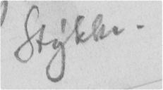Stykke.
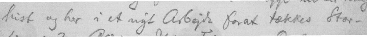hist og her i et nyt Arbejde forat tækkes Stor-
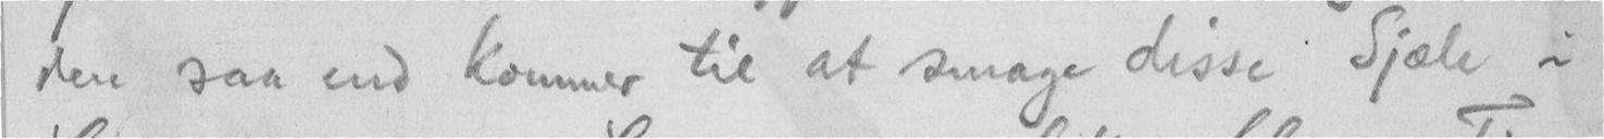den saa end kommer til at smage disse Sjæle i
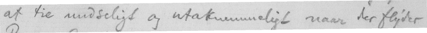at tie undseligt og utaknemmeligt naar der flyder
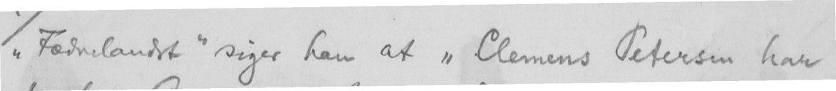"Fædrelandet" siger han at "Clemens Petersen har
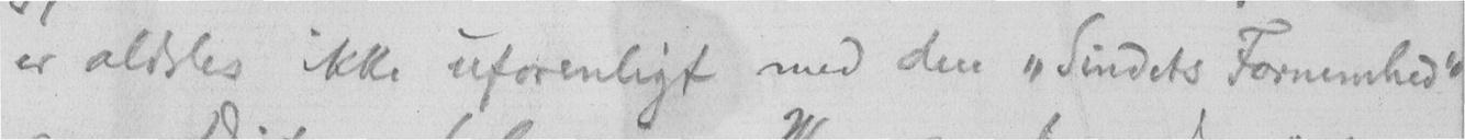er aldeles ikke uforenligt med den "Sindets Fornemhed"
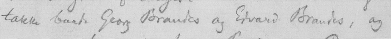takke baade Georg Brandes og Edvard Brandes, og
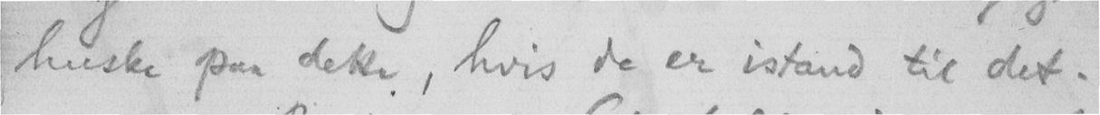huske paa dette, hvis de er istand til det.
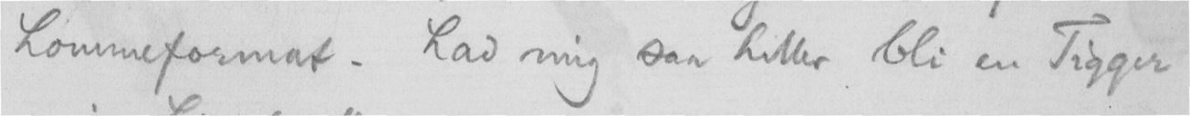Lommeformat. Lad mig saa heller bli en Tigger
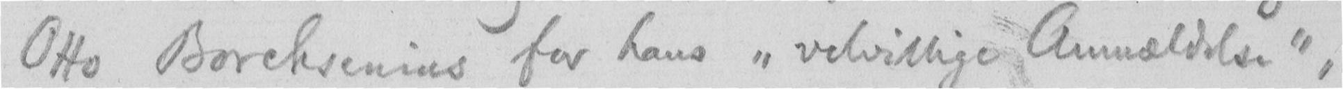Otto Borchsenius for hans "velvillige Anmældelse",
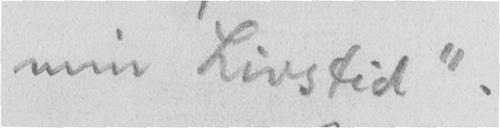min Livstid".
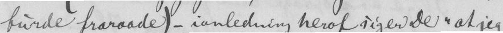burde Fraraade) - ianledning heraf siger De "at jeg
